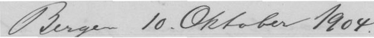Bergen 10. Oktober 1904.
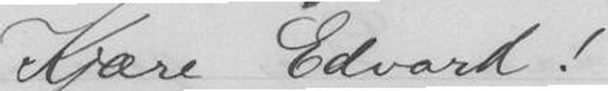Kjære Edvard!
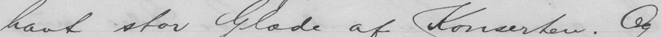havt stor Glæde af Konserten. Og
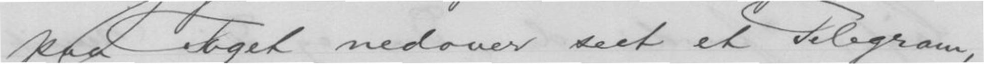paa Toget nedover seet et Telegram,
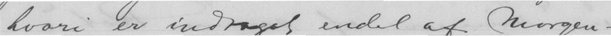hvori er indtaget endel af Morgen-
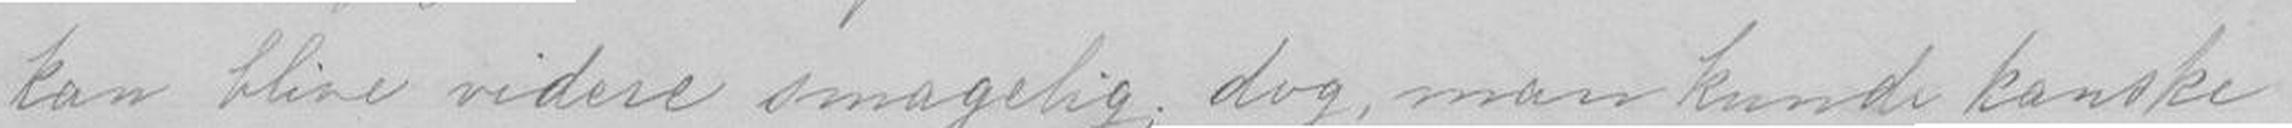kan blive videre smagelig, dog man kunde kanske
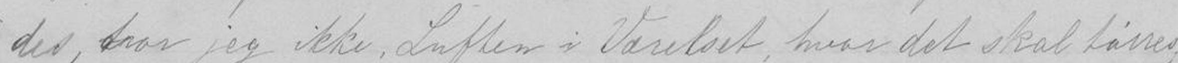des, tror jeg ikke, Luften i Værelset, hvor det skal tømes,
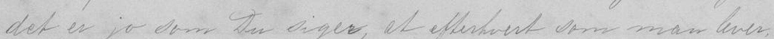det er jo som du siger at efterhvert som man lever
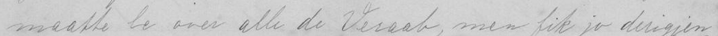maatte le over alle de Veraab, men fik jo derigjen-
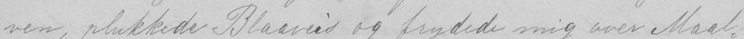gen, plukkede Blaaveis og frydede mig over Maal-
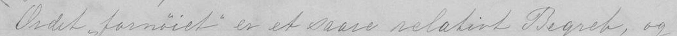Ordet "formøiet" er et saare relativt Begreb, og
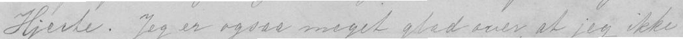Hjerte. Jeg er ogsaa meget glad over, at jeg ikke
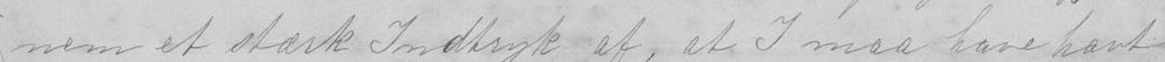nom et stærk Indtryk af, at I maa bare havt
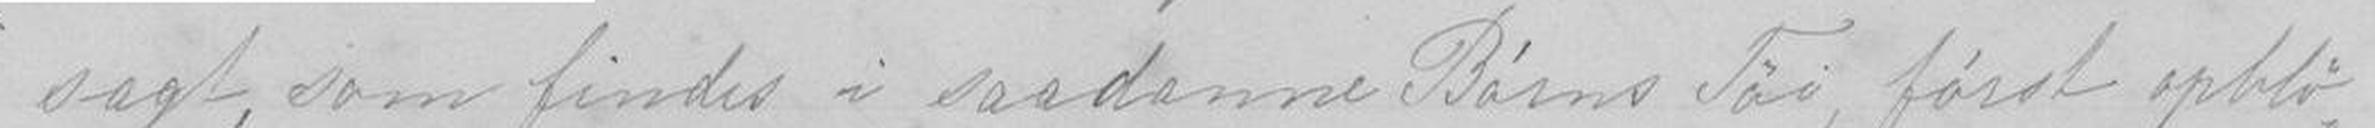sagt, som findes i saadanne Børns Tøi, først opblø-
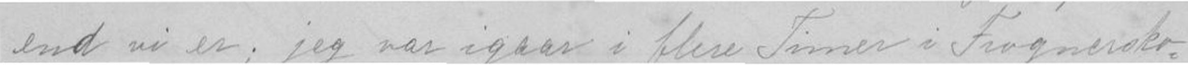end vi er; jeg var igaar i flere timer i Frogneersko-
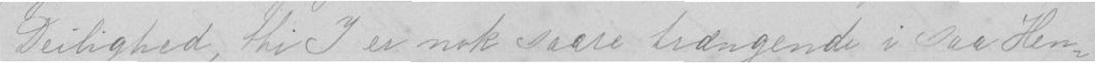Deilighed, thi I er nok saare hængende i saa Hen-
